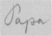Papa
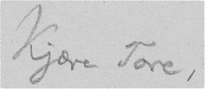Kjære Tore,
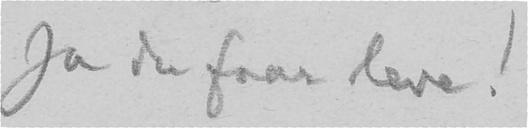Ja du faar leve!
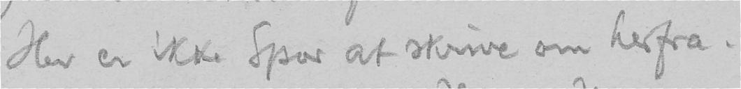Her er ikke Spor at skrive om herfra.
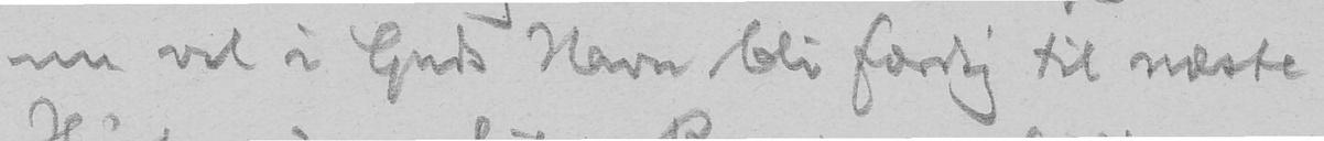nu vel i Guds Navn bli færdig til næste
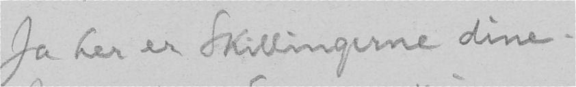Ja her er Skillingerne dine.
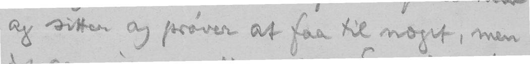og sitter og prøver at faa til noget, men
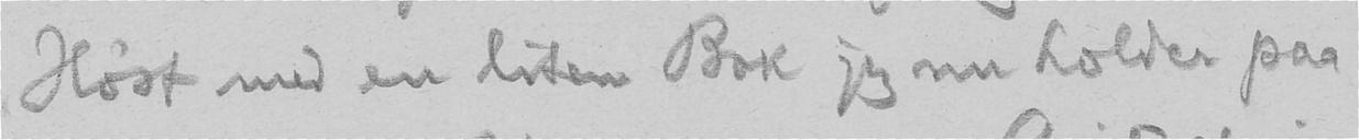Høst med en liten Bok jeg nu holder paa
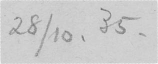28/10. 35
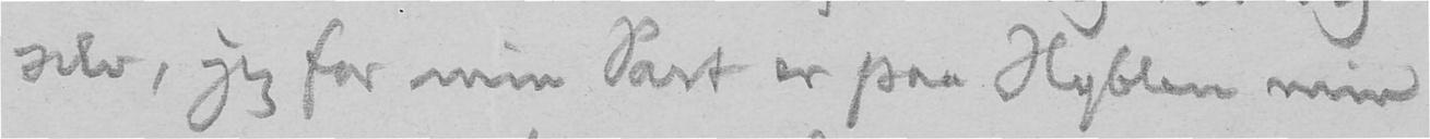selv, jeg for min Part er paa Hyblen min
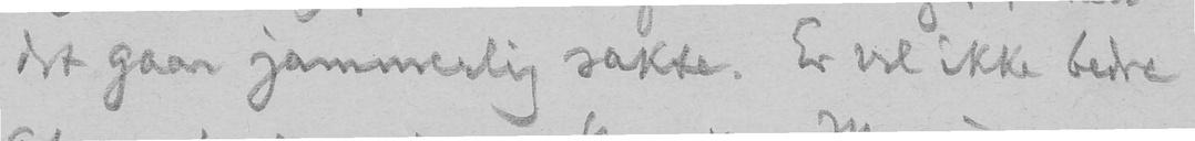det gaar jammerlig sakte. Er vel ikke bedre
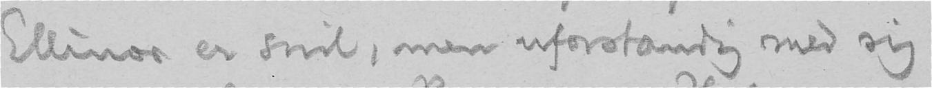Ellinor er snil, men uforstandig med sig
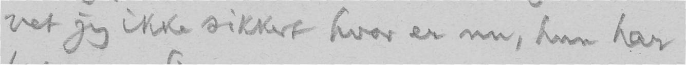vet jeg ikke sikkert hvor er nu, hun har
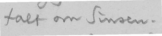talt om Sinsen.
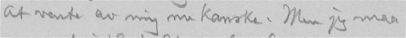at vente av mig nu kanske. Men jeg maa
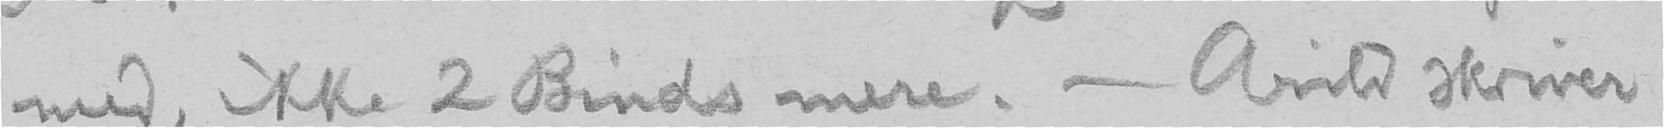med, ikke 2 Binds mere. - Arild skriver
